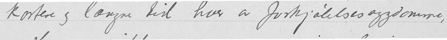kortere og længre tid hver av forkjølelsessygdomme,
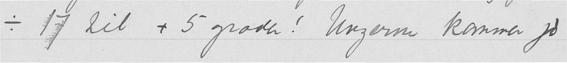-17 til +5 grader! Ungerne kommer jo
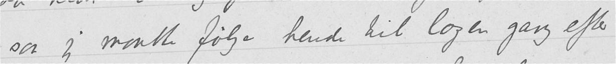saa jeg maatte følge hende til lægen gang efter
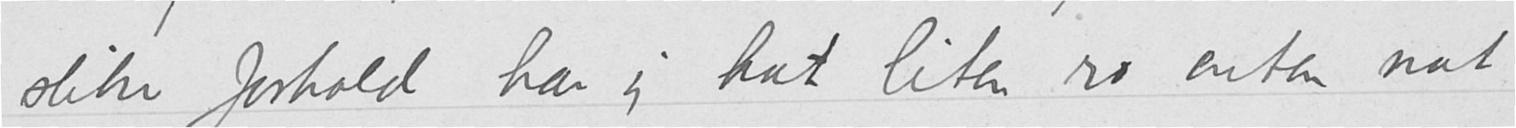slike forhold har jeg hat liten ro enten nat
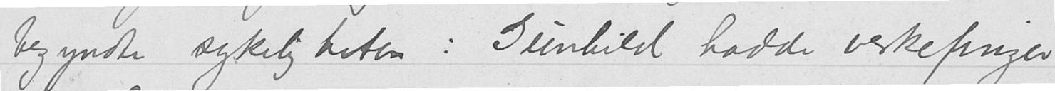begyndte sykeligheten: Gunhild hadde verkefinger
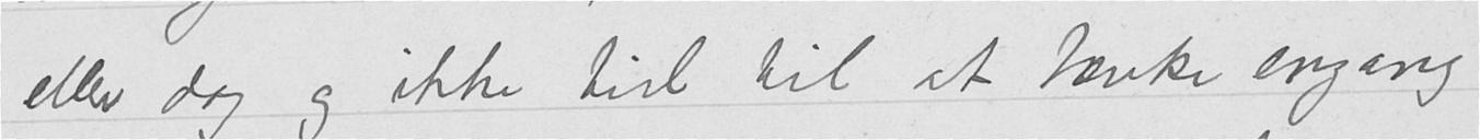eller dag og ikke tid til at tænke engang
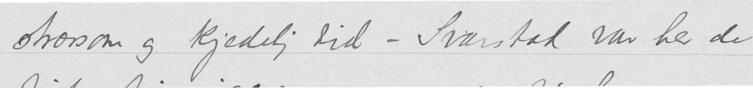strævsom og kjedelig tid – Svarstad var her de
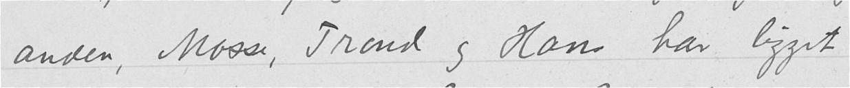anden, Mosse, Trond og Hans har ligget
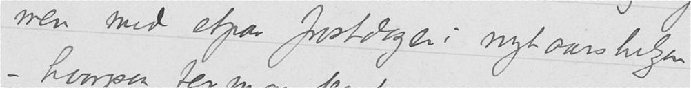men med etpar frostdager i nytaarshelgen
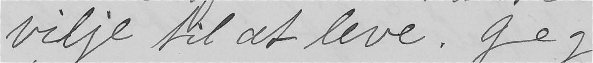vilje til at leve. Jeg
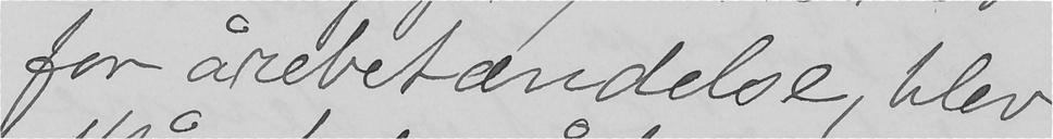for årebetændelse, blev
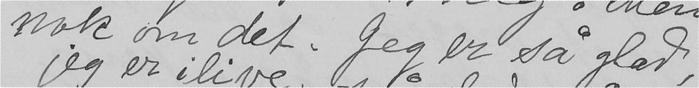nok om det. Jeg er så glad,
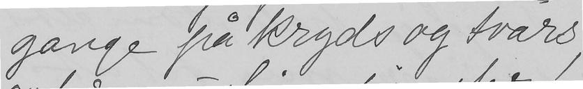gange på kryds og tværs,
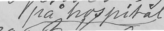på hospital
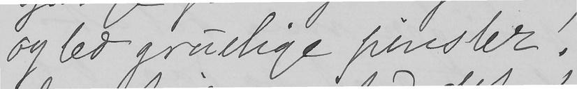og led gruelige pinsler.
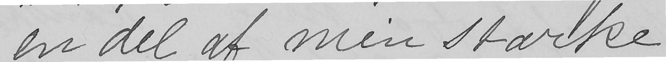en del af min stærke
